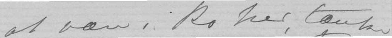at være i Ro her, tænker
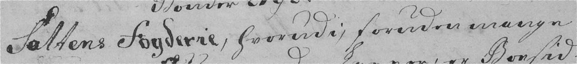Saltens Fogderie, hvorudi, foruden mange
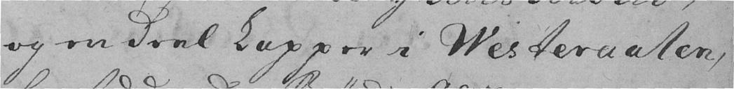og en deel Lapper i Westeraalen,
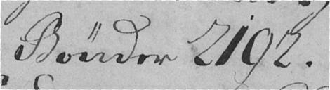Bønder 2192.
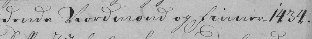dende Nordmænd og Finner 1434.
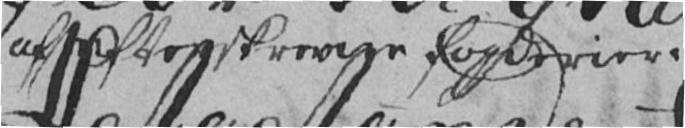af orskrevige fogderier.
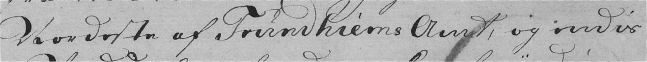Nordeste af Trundhiems Amt, og endes
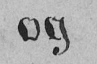og
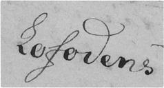Lofodens
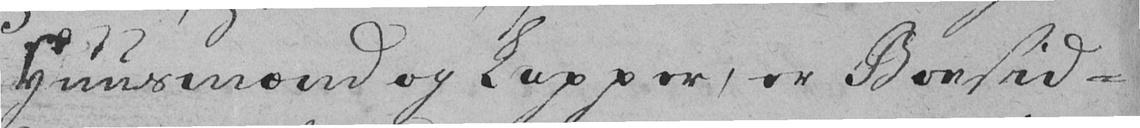Huusmænd og Lapper, er Boesid-
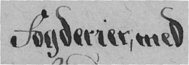Fogderier med
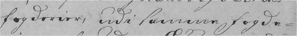Fogderier, udi samme Fogde-
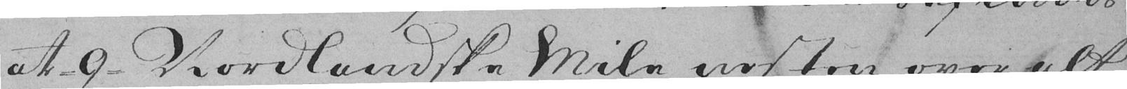at 9 Nordlandske Mile nesten over alt
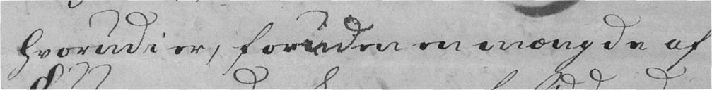hvorudi er, foruden en mængde af
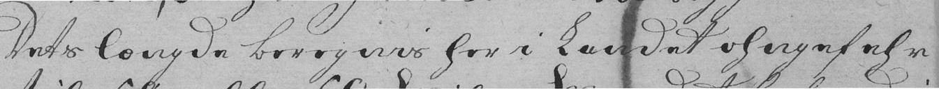Dets længde beregnis her i Landet ohngefehr
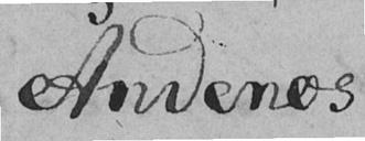Andenæs
